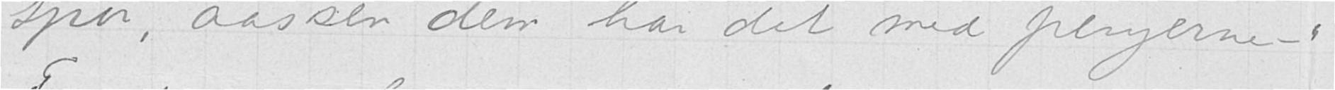spør, aassen dem har det med pengerne-"
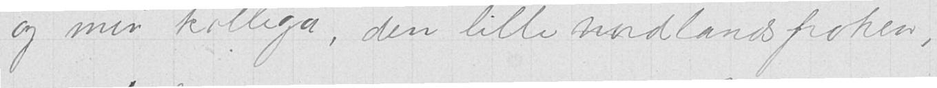og min kollega, den lille nordlandsfrøken,
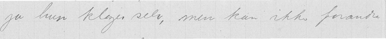ja hun klager selv, men kan ikke forandre
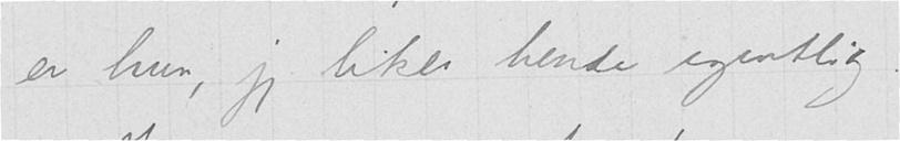er hun, jeg liker hende egentlig.
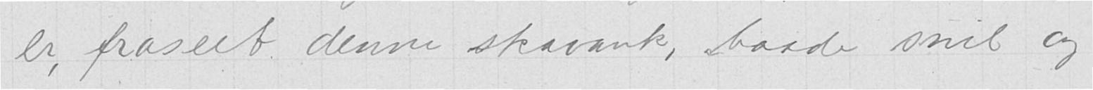er fraseet denne skavank, baade snil og
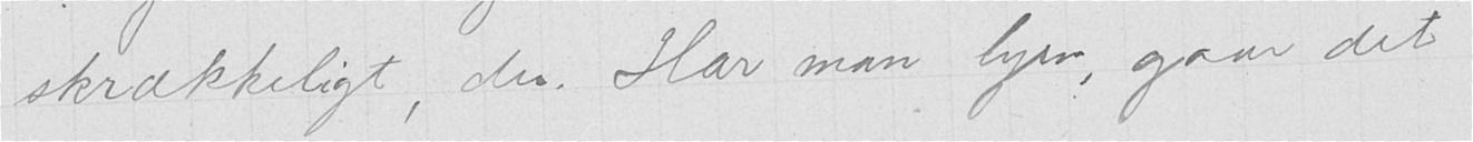skrækkeligt, du. Har man hjem, gaar det
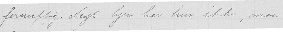fornuftig. Noget hjem har hun ikke, maa
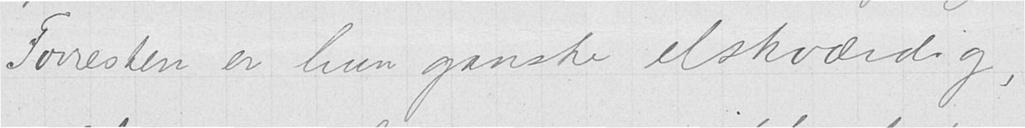Foresten er hun ganske elskværdig,
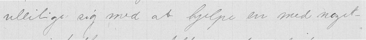uleilige sig med at hjelpe en med noget -
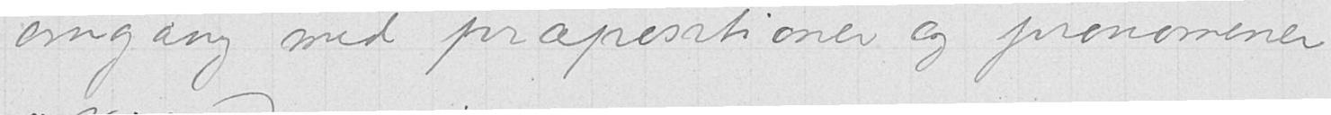omgang med præpositioner og pronomener
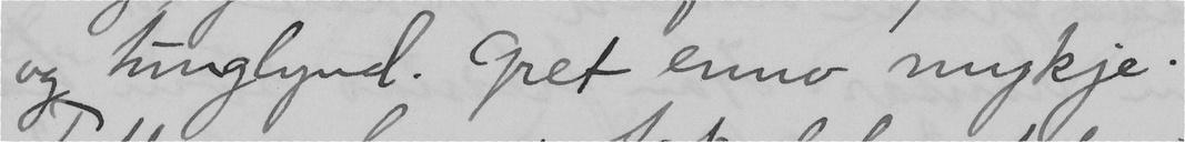og tunglynd. Gret enno mykje.
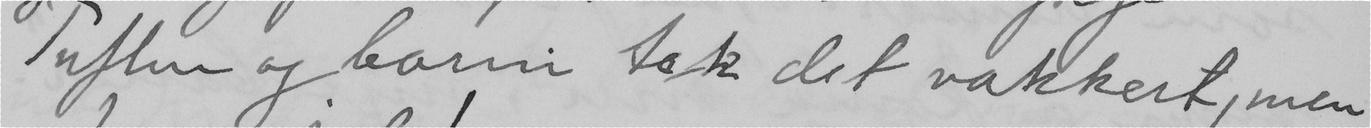Tuften og barni tek det vakkert, men
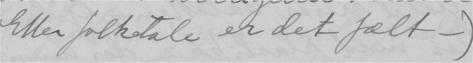Etter folketale er det fælt.
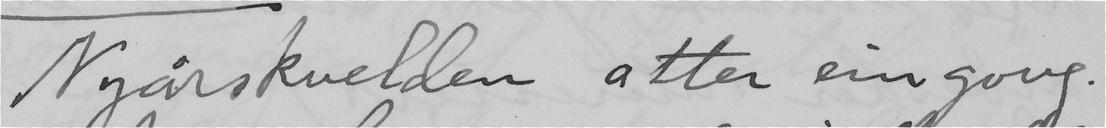Nyårskvelden atter ein gong.
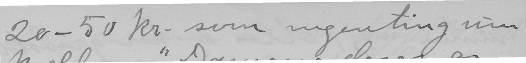20-50 kr. som ingenting um
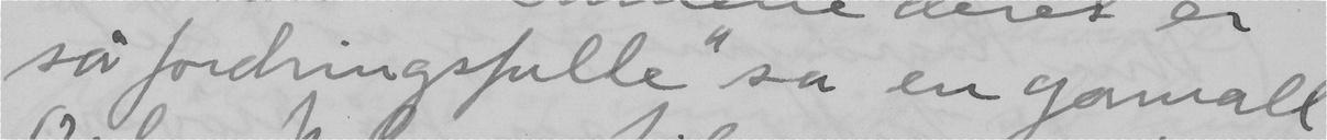så fordringsfulle" sa en gamall
